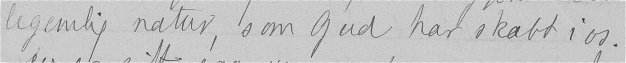legemlig natur, som Gud har skabt i os.
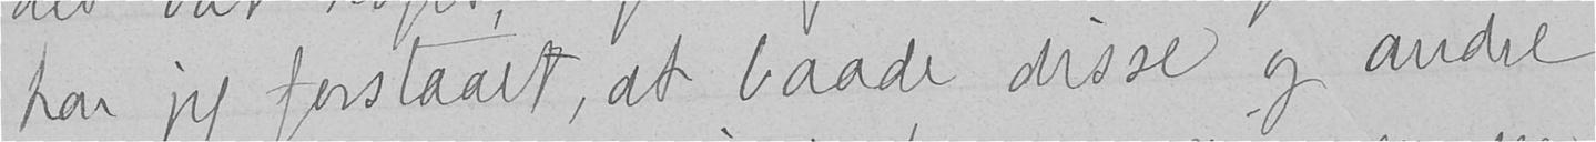har jeg forstaaet, at baade disse og andre
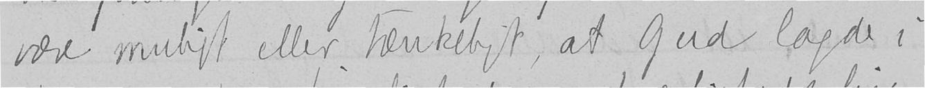være muligt eller tænkeligt, at Gud lagde i
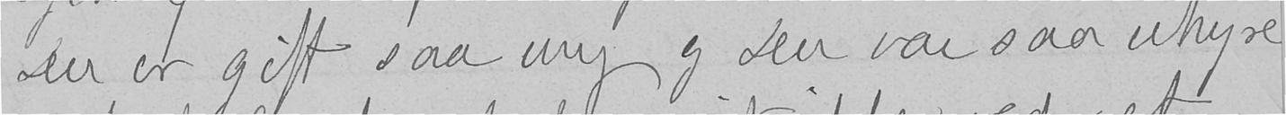Du er gift saa ung og Du var saa uhyre
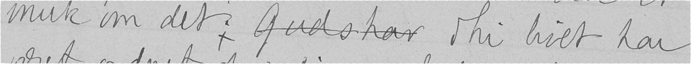muk om det. Gudshar Thi livet har
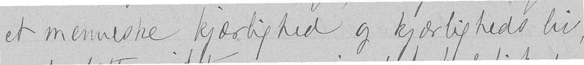et menneske kjærlighed og kjærligheds liv,
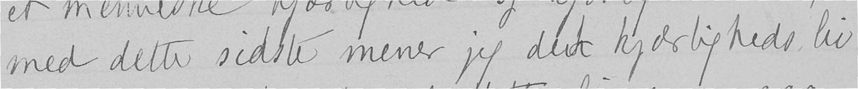med dette sidste mener jeg den kjærligheds liv
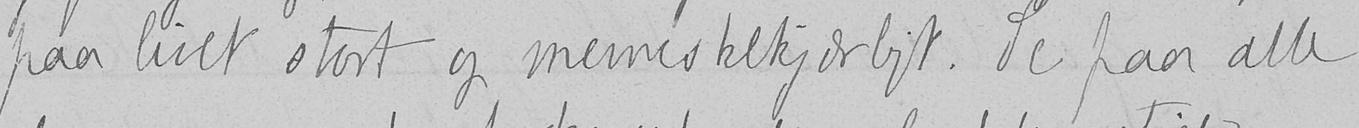paa livet stort og mennskekjærligt. Se paa alle
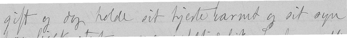gift og dog holde sit hjerte varmt og sit syn
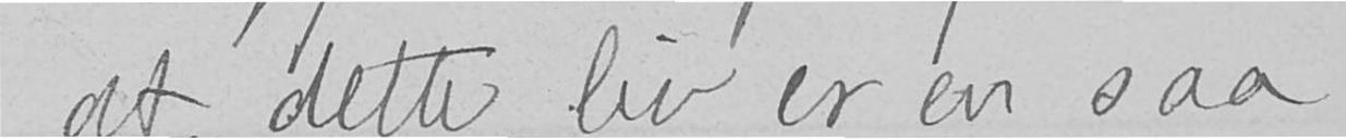at dette liv er en saa
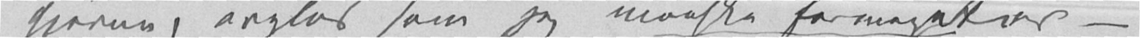gjerne, orgløs som jeg maaske for meget er –
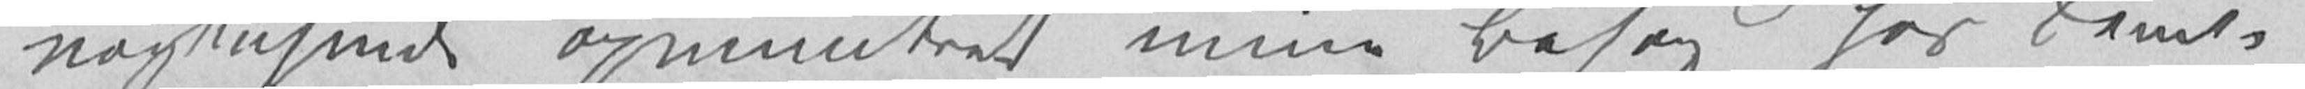nogensinde opmuntret mine Besøg hos Dem,
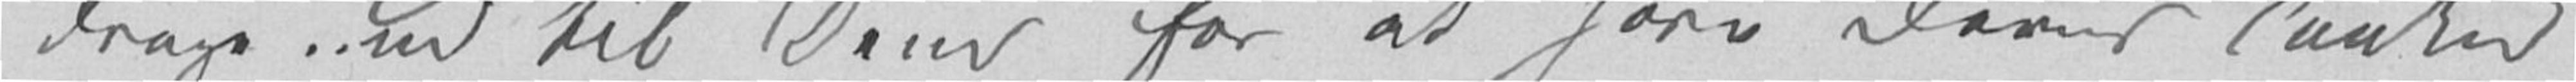drage ud til Dem for at høre Deres Tanker
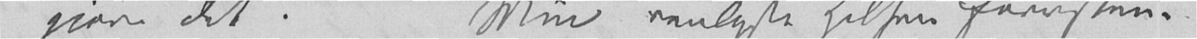gjøre det. Min venligste Hilsen forresten.
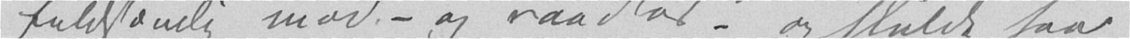fuldstændig modog raadløs – og skulde saa
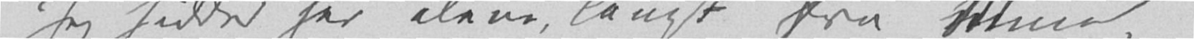Jeg sidder her alene, langt fra Mine,
